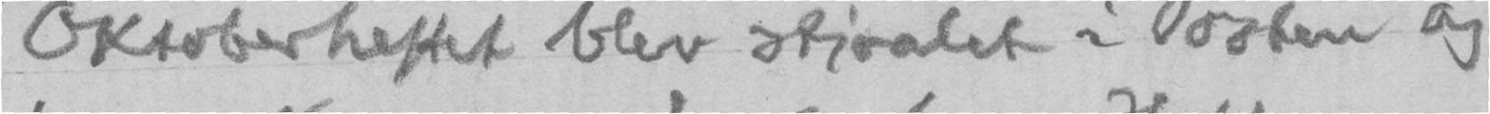Oktoberheftet blev stjaalet i Posten og
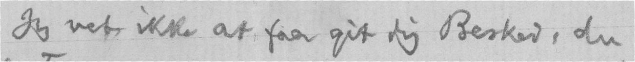Jeg vet ikke at faa git dig Besked, du
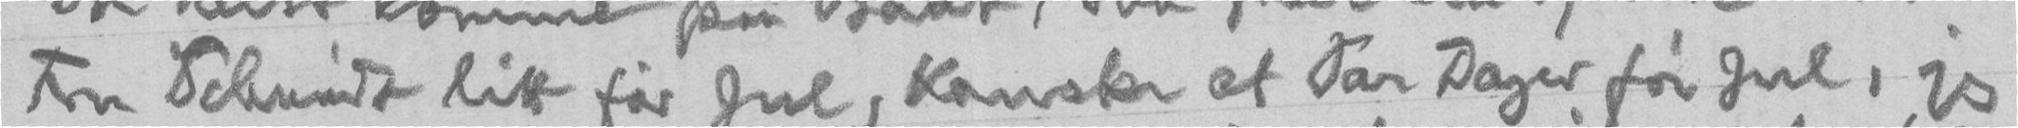Fru Schmidt litt før Jul, kanske et Par Dager før Jul, jeg
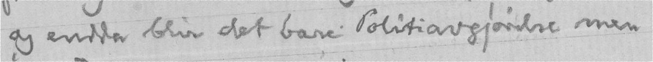og endda blir det bare Politiavgjørelse men
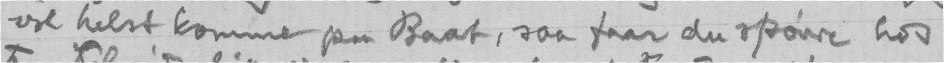vil helst komme paa Baat, saa faar du spørre hos
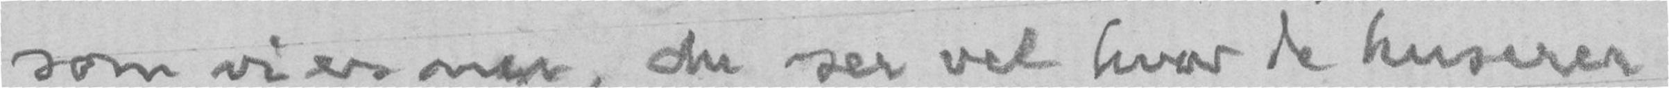som vi er nu, du ser vel hvor de huserer
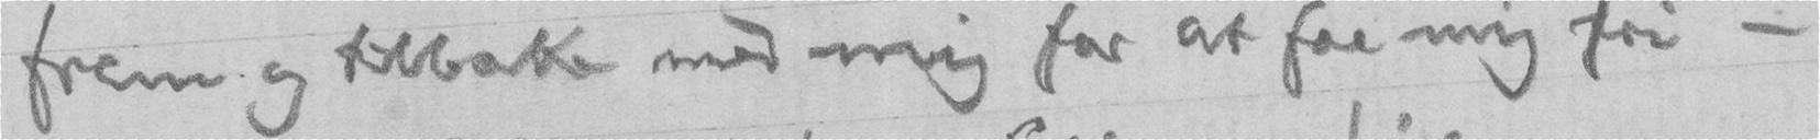frem og tilbake med mig for at faa mig fri -
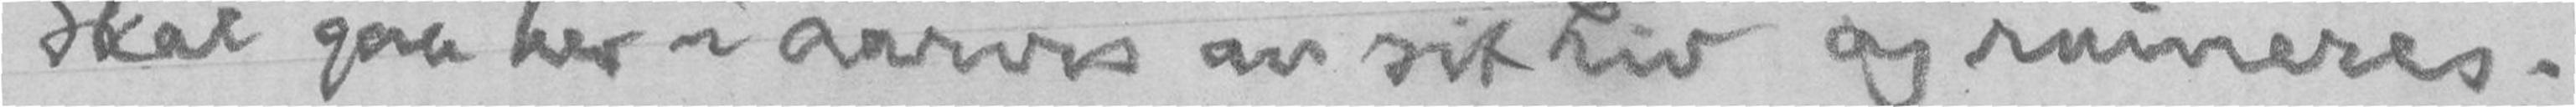skal gaa her i aarevis av sit Liv og ruineres.
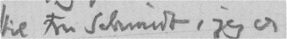til Fru Schmidt, jeg er
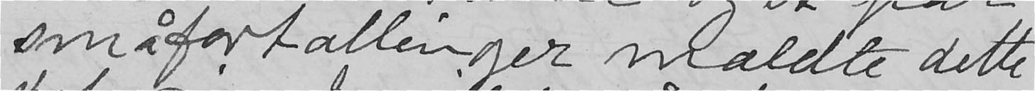småfortællinger mældte dette
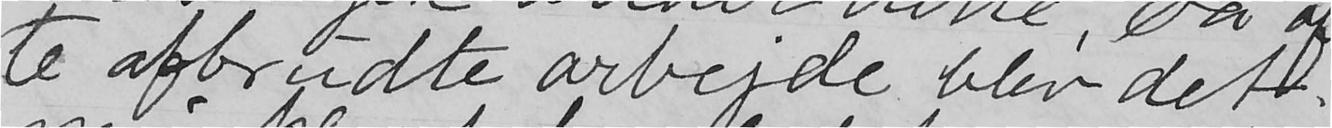te afbrudte arbejde blev det
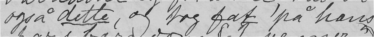også dette, og tog fat på hans
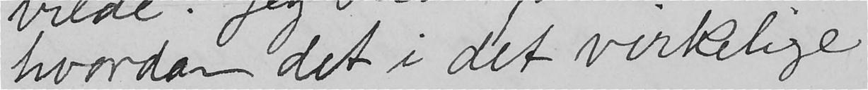hvordan det i det virkelige
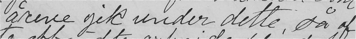årene gik under dette, så of-
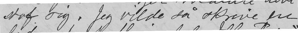stof sig. Jeg vilde så skrive en
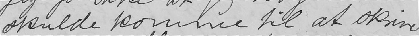skulde komme til at skrive
